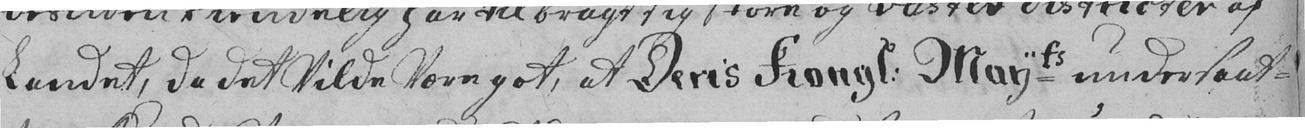Landet, da det Vilde Være got, at Deris Kongl. Mayts undersaat-
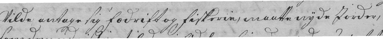Vilde antage sig Fædrift og Fiskerie, maatte nyde Jorder,
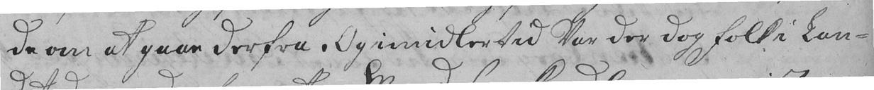de om at gaae derfra. Og imidlertid Var der dog folk i Lan-
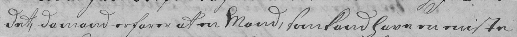det, da mand erfarer at en Mand, som kand have en eniste
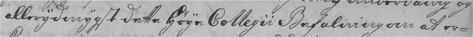allerydmygst dette Høye Collegii Befalning om at er-
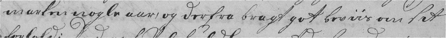marken nogle aar, og derfra bragt got beviis om sit
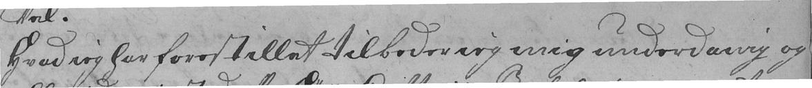Hvad ieg har forestillet tilbeder ieg mig underdanig og
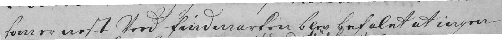som er nest Veed Findmarken blev befalet at ingen
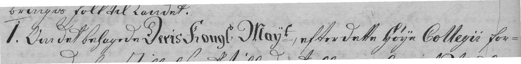1. Om det behagede Deris Kongl. Mayt, efter dette Høye Collegii for-
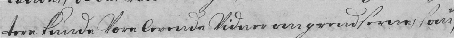tere kunde Være levende Vidner om grendserne, som
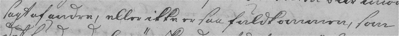sagt af andre, eller ikke er saa fuldkommen, som
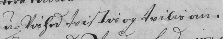u-Vished tvistis og tvilis om.
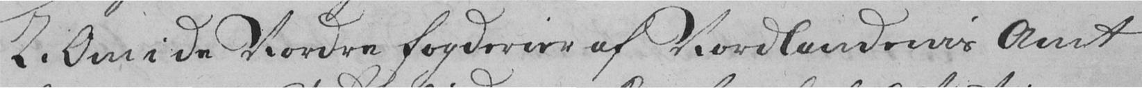2. Om i de Nordre Fogderier af Nordlandenis Amt
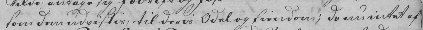som den udvistis, til deris Odel og Eiendom; da nu intet af
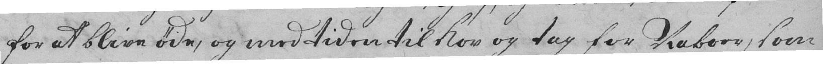for at blive øde, og med tiden til Kov og tag for Naboer, som
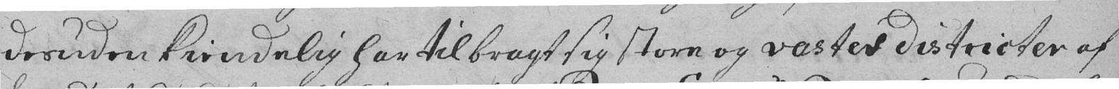desuden kiendelig har tilbragt sig store og vaster districter af
